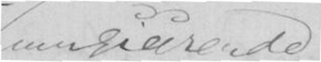musicerende
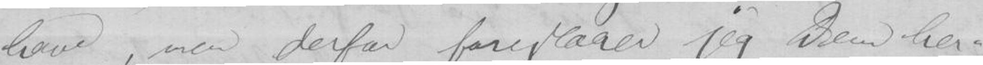have, men derfor foreslaaer jeg Dem her-
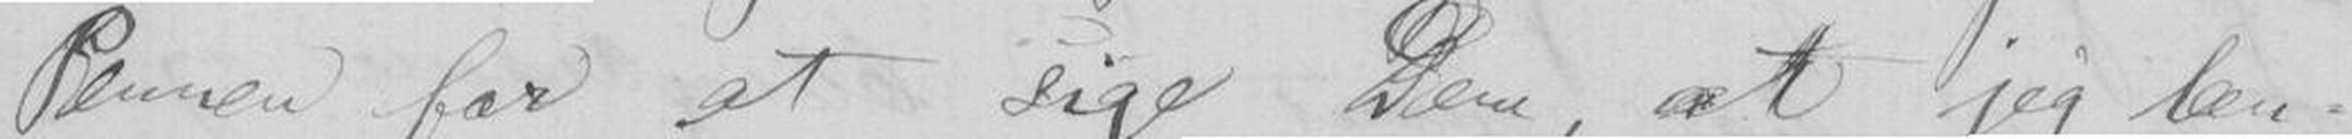Pænnen for at sige Dem, at jeg læn-
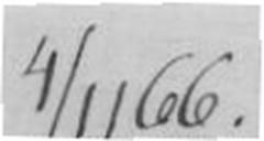4/11 66
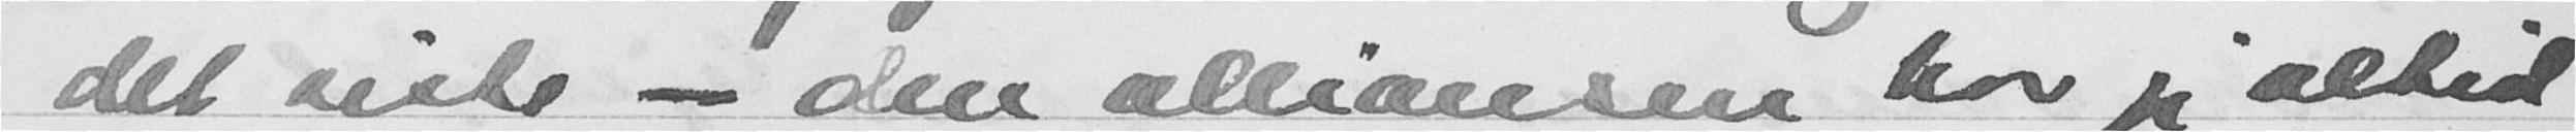det siste - den alliansen har jeg altid
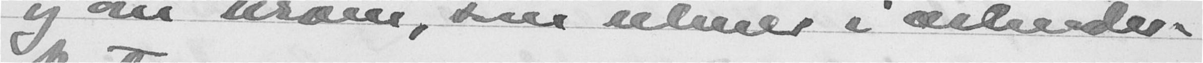og om uroen, som ulmner i arbeider-
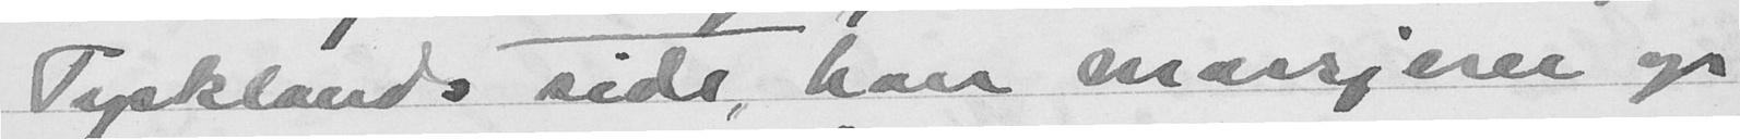Tyklands side han marsjerer op
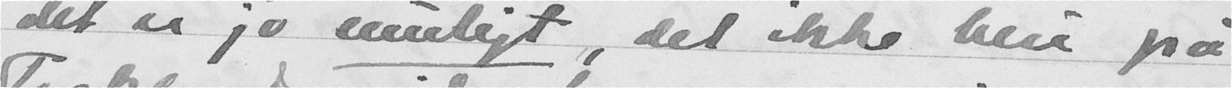det er jo muligt, det ikke blir på
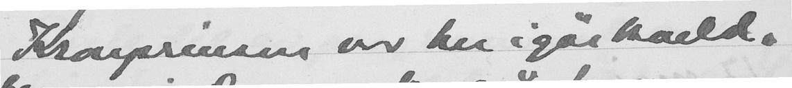Kronprinsen var her igårkveld.
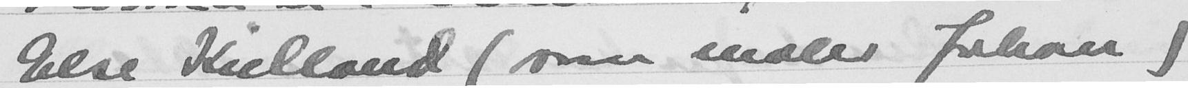Else Kielland (som maler Johan)
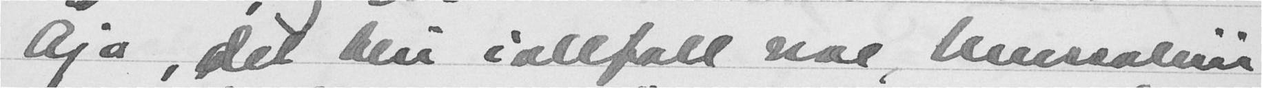Aja, det blir iallfall noe, Mussolini
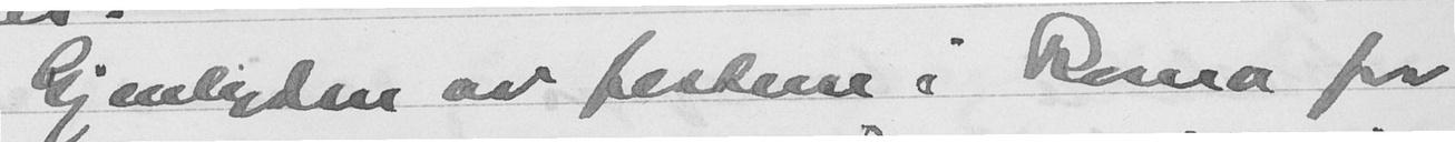Gjenlyden av festene i Roma for
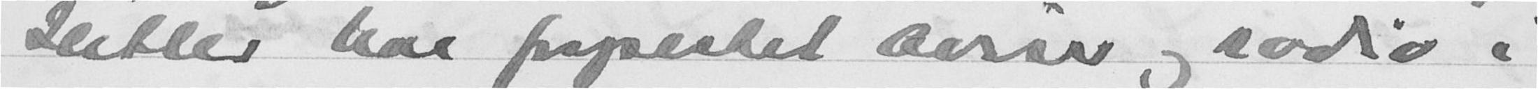Hitler har forpestet aviser og radio i
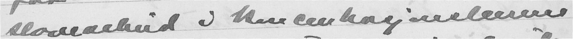slavearbeid i konsentrasjonsleirene
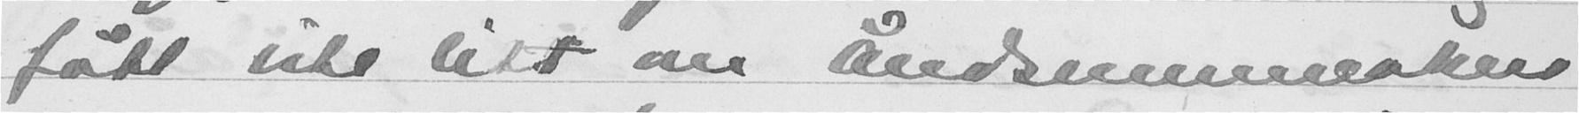fått vite litt om åndsmenneskers
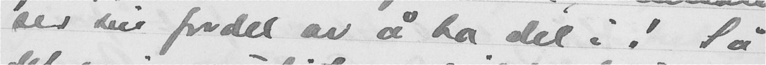ser nu fordel av å ha del i! Så
